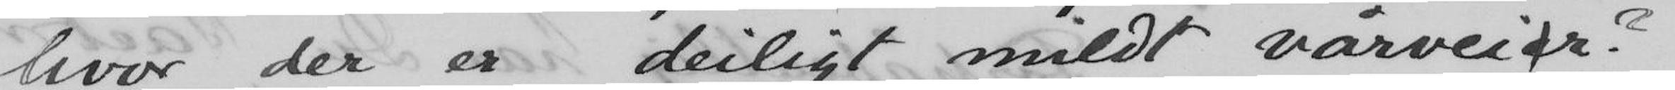hvor der er deiligt mildt vårveir?
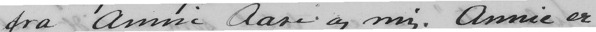fra Annie Aase og mig. Annie er
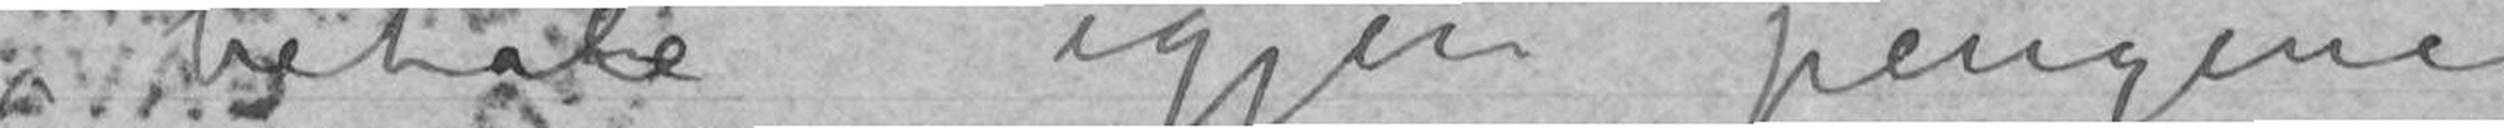betale igjen pengene
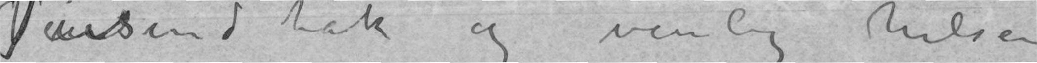Tusind tak og venlig hilsen
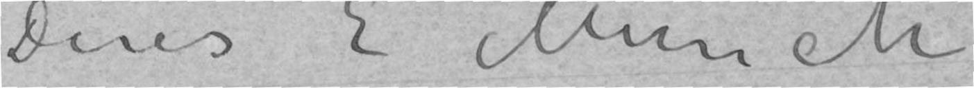Deres E Munch
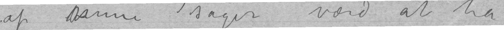af mine sager værd at ha –
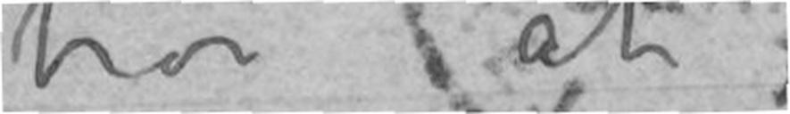tror at
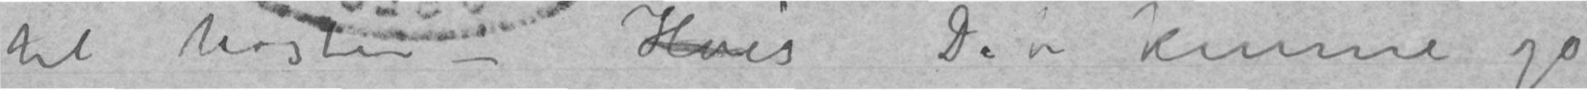til høsten – Hvis Det kunne jo
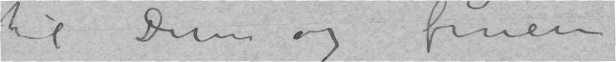til Dem og fruen
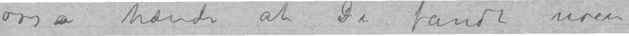osså hænde at De fandt noen
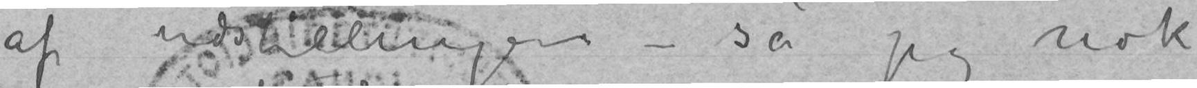af udstillingen – så jeg nok
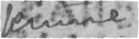kunne
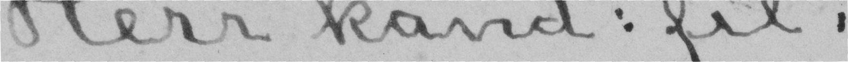Herr kand: fil:
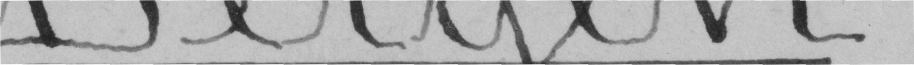Bergen.
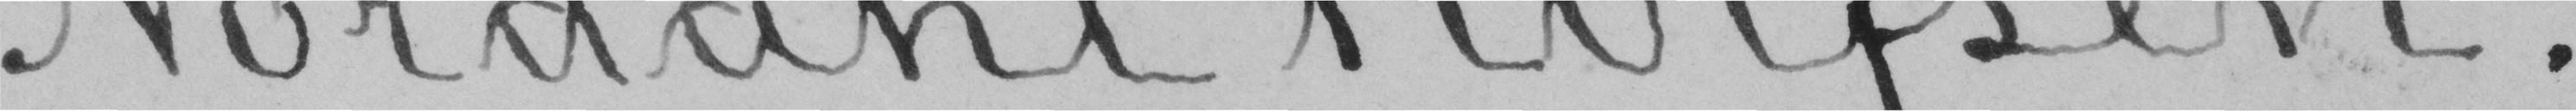Nordahl Rolfsen.
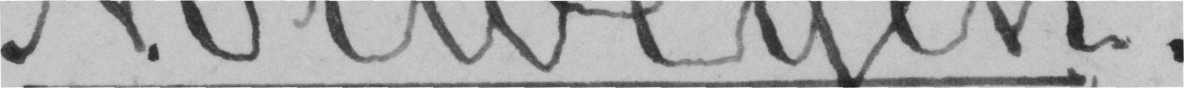Norwegen.
